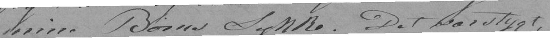mine Børns Lykke. Det var stygt,
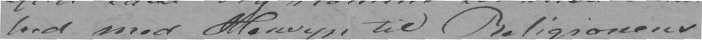hed med Hensyn til Religionens
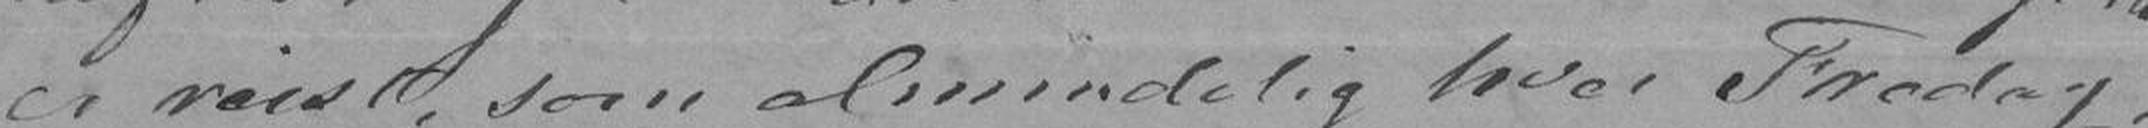er reist, som almindelig hver Fredag,
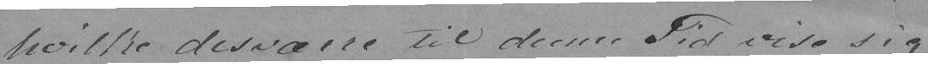hvilke desværre til denne Tid vise sig
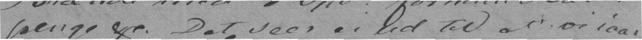penge ogc. Det seer ei ud til at vi iaar
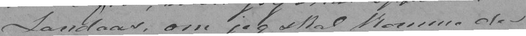Landaas, om jeg skal komme der
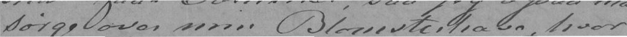sørge over min Blomsterhave, hvor
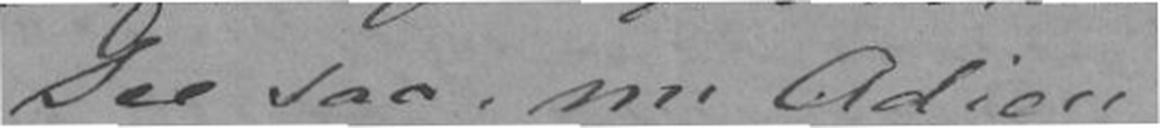see saa, nu Adieu
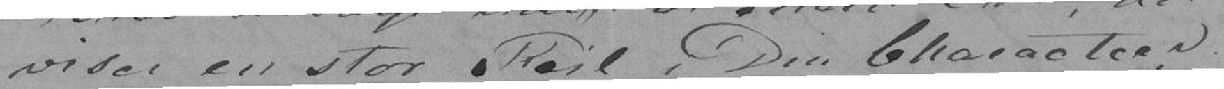viser en stor Feil i Din Characteer.
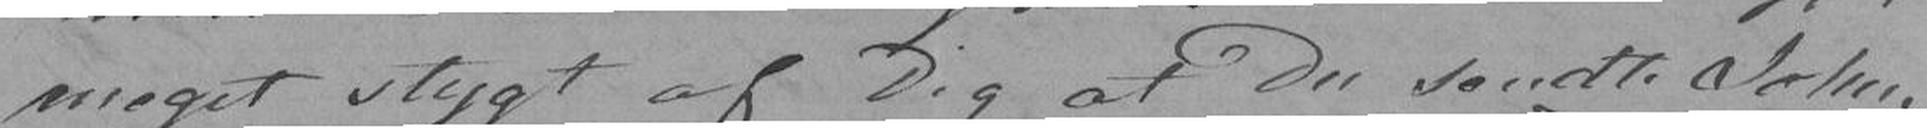meget stygt af Dig at Du sendte Johns
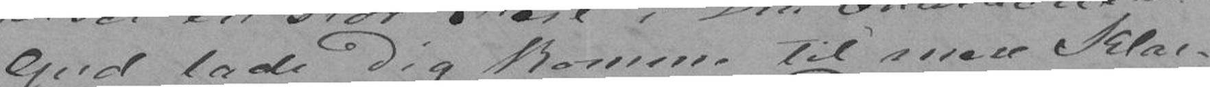Gud lade Dig komme til mere Klar-
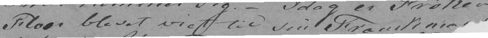Floer blevet viet til sin Franskma
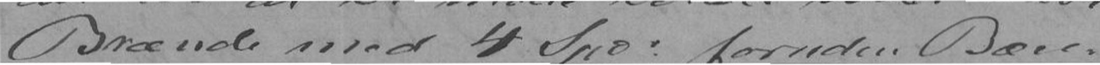Brænde med 4 Spd. foruden Bære-
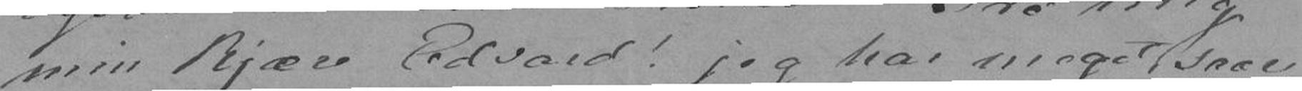min kjære Edvard! jeg har meget, saae
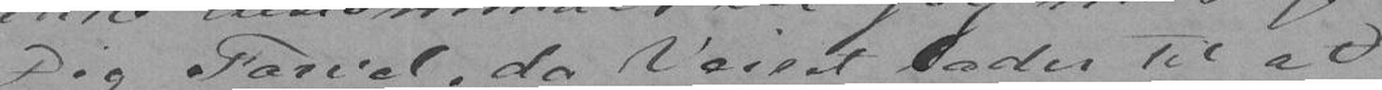Dig Farvel, da Veiret lader til at
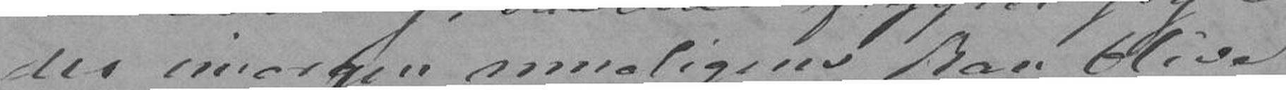der imorgen mueligens kan blive
